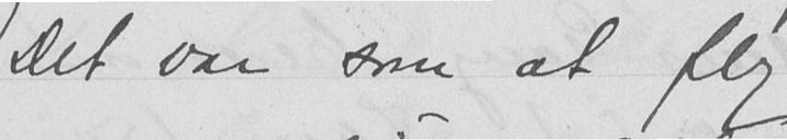Det var som at fly
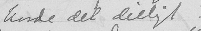havde det deiligt.
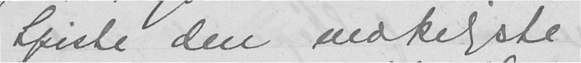Spiste den makeligste
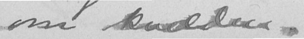om kvelden.
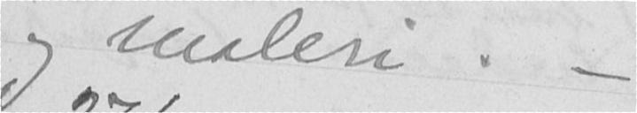og maleri. -
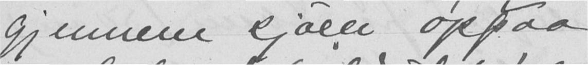gjennem sjøen oppaa
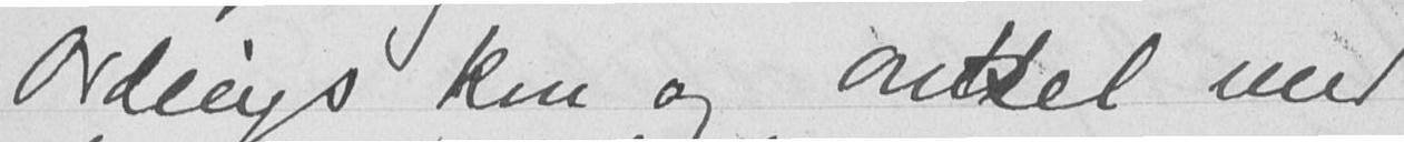Ordings kom og onkel med
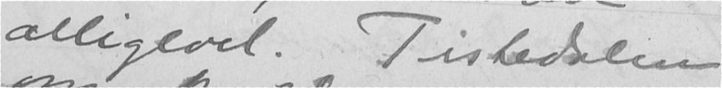alligevel. Tistedalen
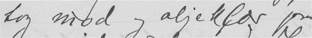tog mod og oljeklær paa
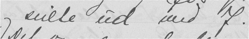og seilte ud med J.
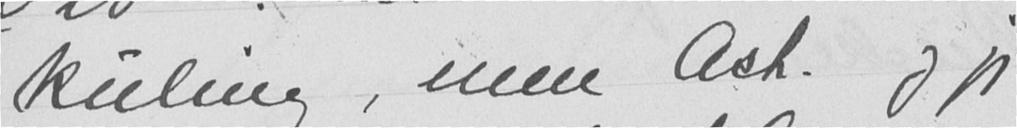kuling, men Astr. og jeg
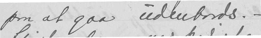paa at gaa udenbords. -
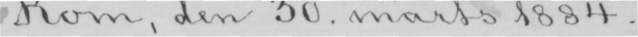Rom, den 30. marts 1884.
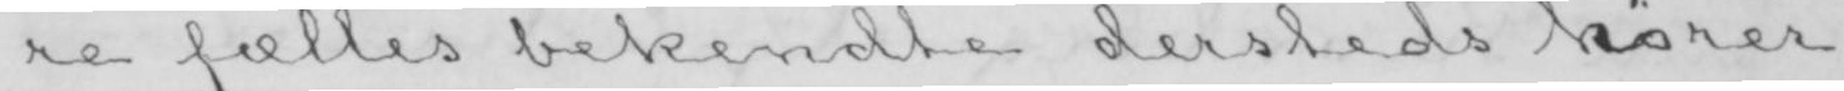re fælles bekendte dersteds hører
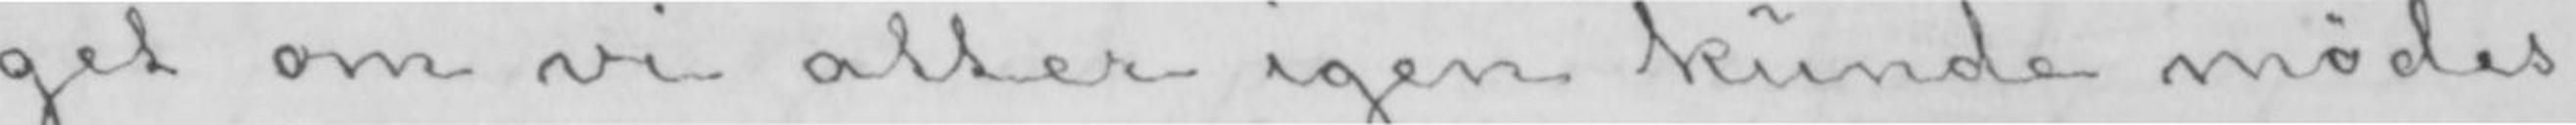get om vi atter igen kunde mødes
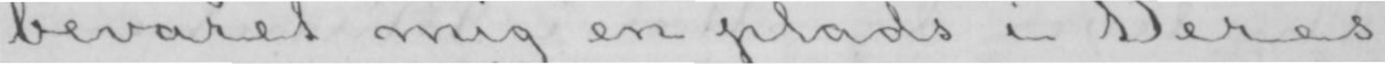bevaret mig en plads i Deres
